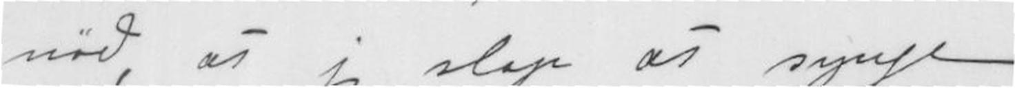nød, at jeg slap at synge
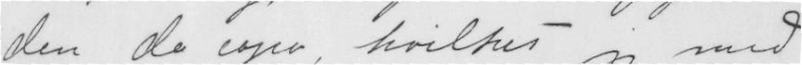den da capo, hvilket jeg med
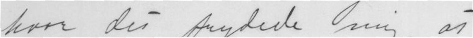hvor det frydede mig at
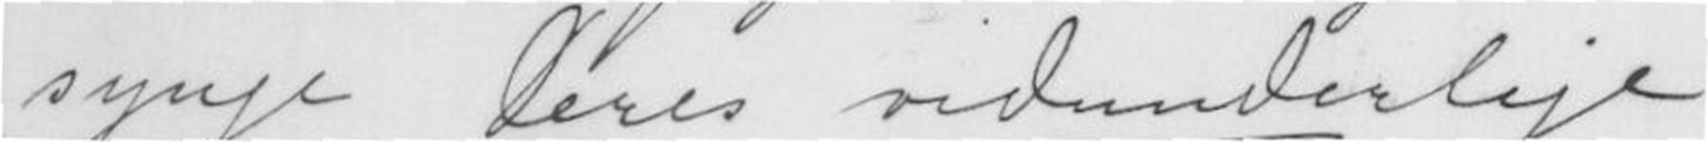synge Deres vidunderlige
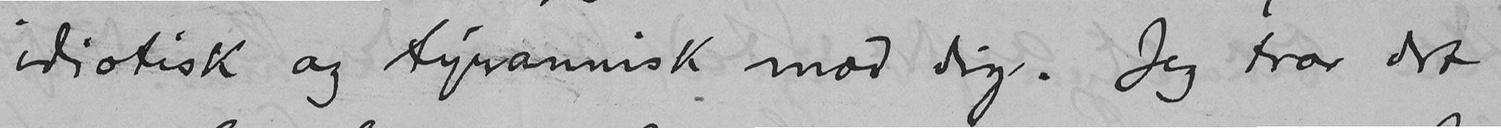idiotisk og tyrannisk mod dig. Jeg tror det
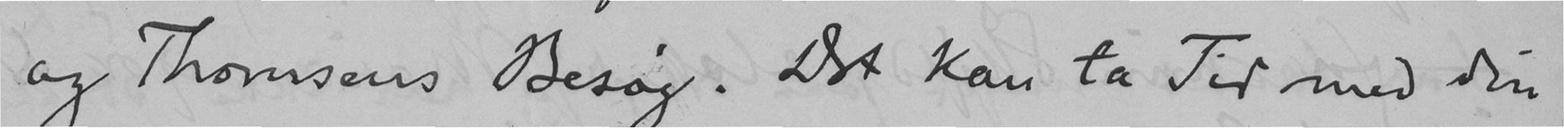og Thomsens Besøg. Det kan ta Tid med din
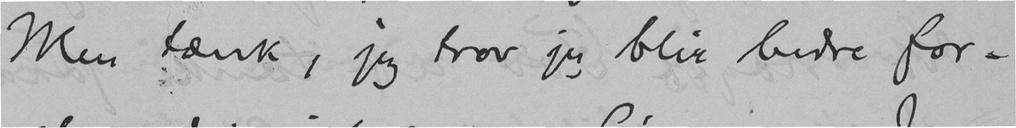Men tænk, jeg tror jeg blir bedre for-
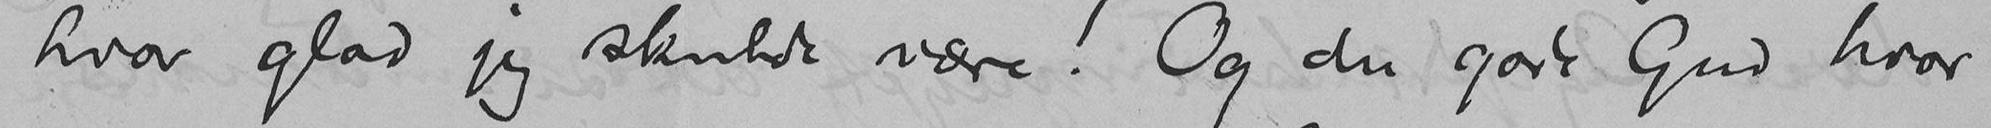hvor glad jeg skulde være! Og du gode Gud, hvor
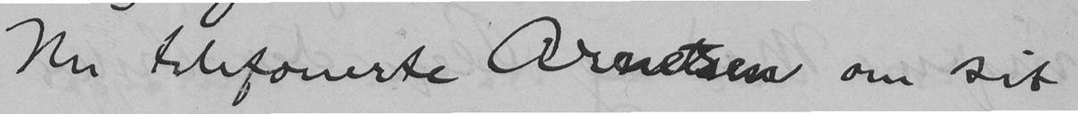Nu telefonerte Arnesen om sit
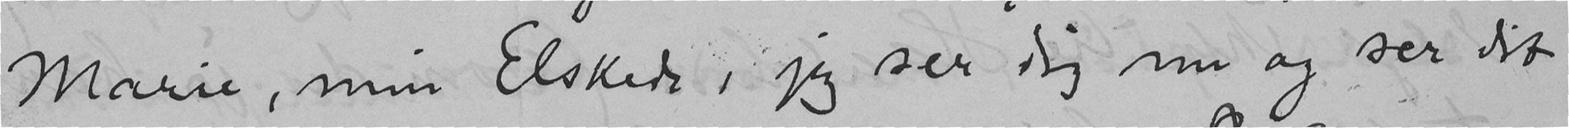Marie, min Elskede, jeg ser dig nu og ser dit
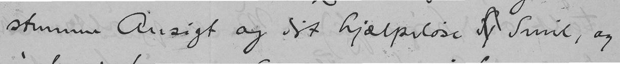stumme Ansigt og dit hjælpeløse fl Smil, og
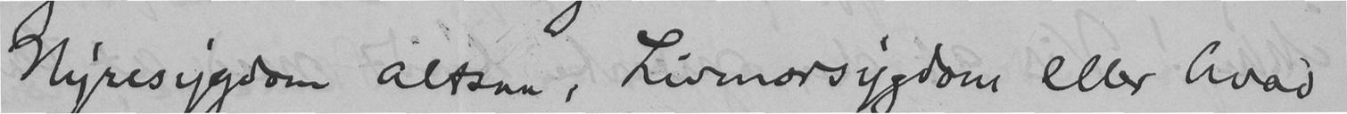Nyresygdom altsaa, Livmorsygdom eller hvad
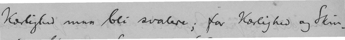Kærlighed maa bli svalere; for Kærlighed og Skin-
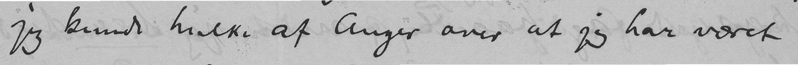jeg kunde hulke af Anger over at jeg har været
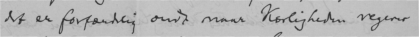det er forfærdelig ondt naar Kærligheden regerer
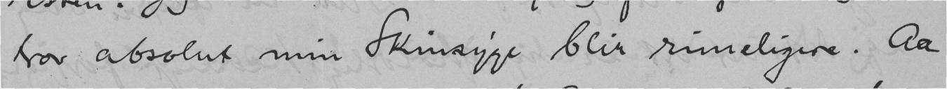tror absolut min Skinsyge blir rimeligere. Aa
